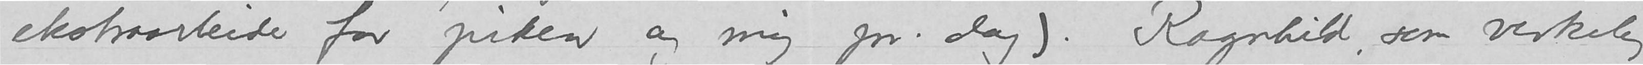ekstraarbeide for piken og mig pr. dag). Ragnhild som virkelig
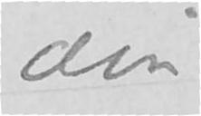din
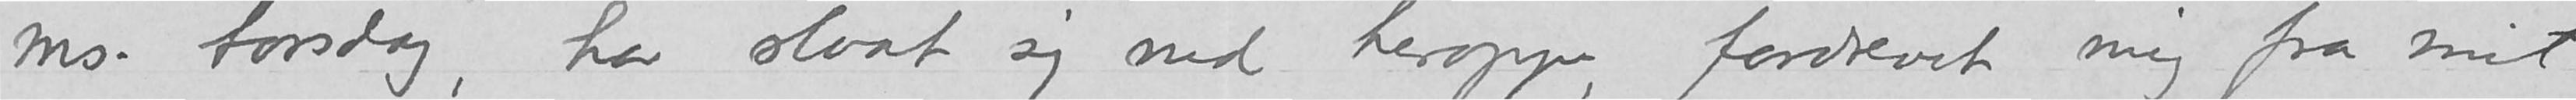ms. torsdag, har slaat sig ned heroppe, fordrevet mig fra mit
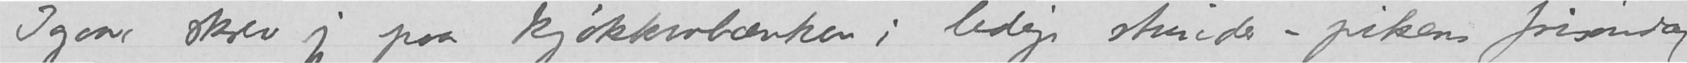Igaar skrev jeg paa kjøkkenbænken i ledige stunder – pikens frisøndag
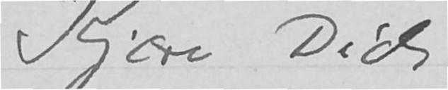Kjære Dichi/Didi
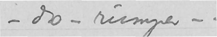– do – rumper –.
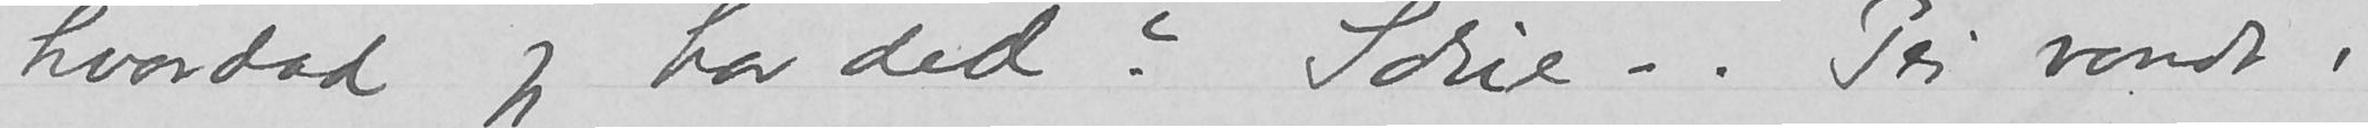Hvordad jeg har ded? Schie –. Pei vondt i
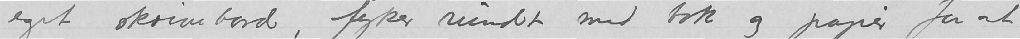eget skrivebord, fyker rundt med bok og papir for at
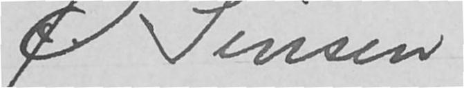Ø. Sinsen
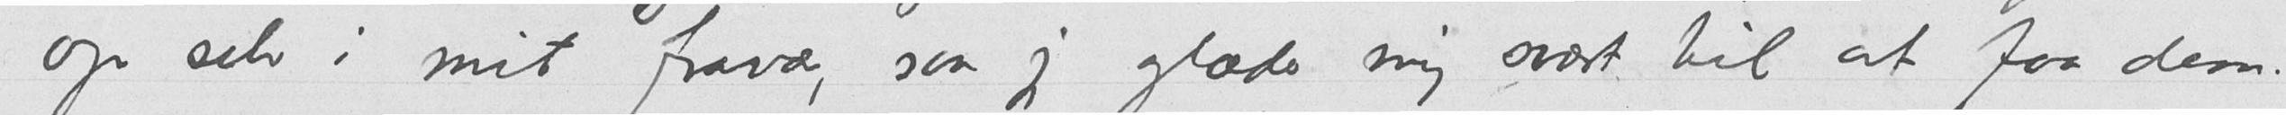op selv i mit fravær, saa jeg glæder mig svært til at faa dem.
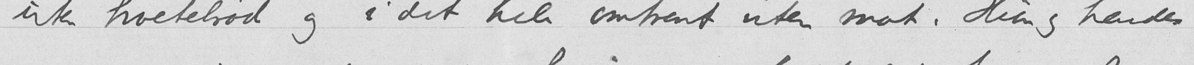uten hvetebrød og i det hele omtrent uten mat. Hun og hendes
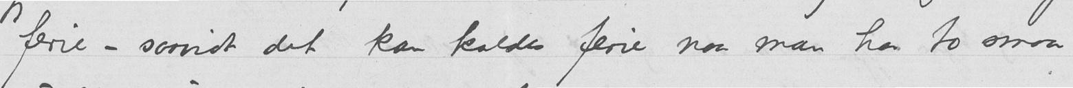ferie – saavidt det kan kaldes ferie naar man har to smaa,
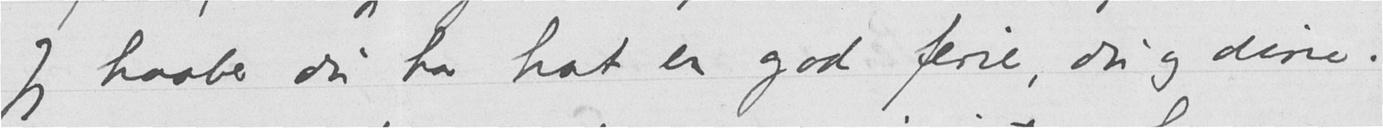Jeg haaber du har hat en god ferie, du og dine.
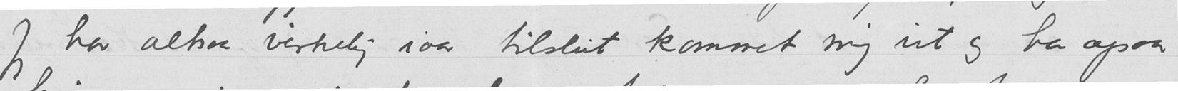Jeg har altsaa virkelig iaar tilslut kommet mig ut og har ogsaa
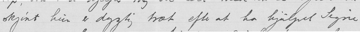- skjønt hun er dygtig træt efter at ha hjulpet Signe
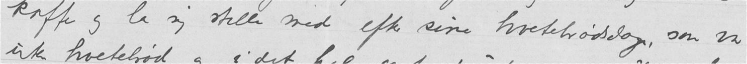kaffe og la sig stelle med efter sine hvetebrødsdags, som var
